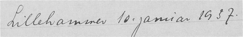Lillehammer 10. januar 1937.
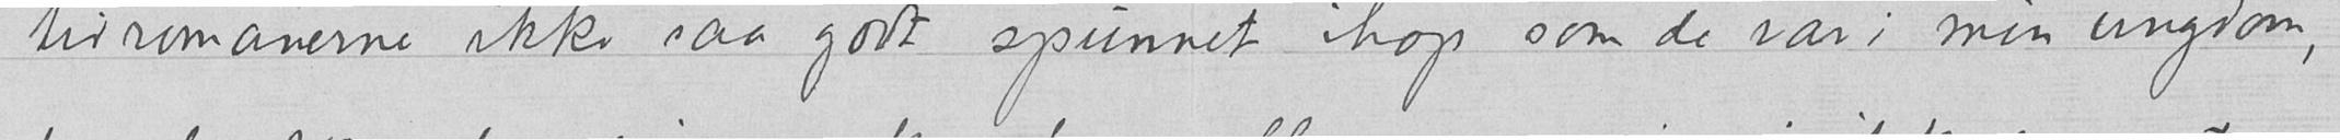tivromanerne ikke saa godt spunnet ihop som de var i min ungdom,
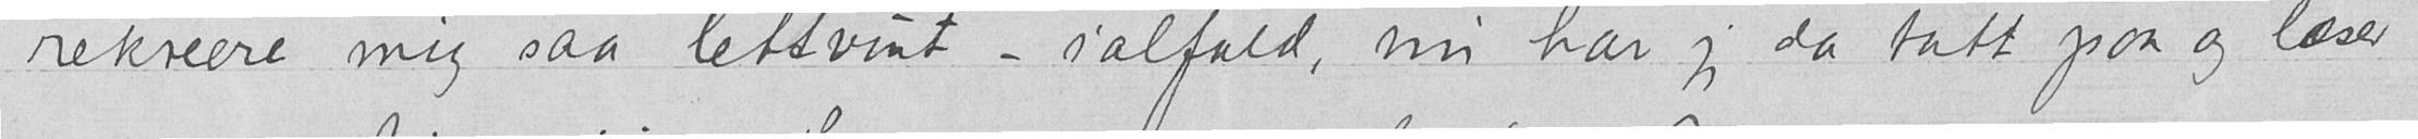rekreere mig saa lettvint – ialfald, nu har jeg da tatt paa og læser
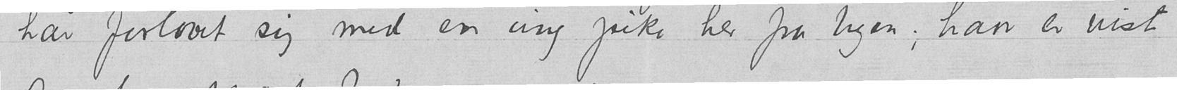har forlovet sig med en ung pike her fra byen; han er vist
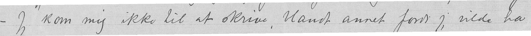– Jeg kom mig ikke til at skrive, blandt annet fordi jeg vilde ha
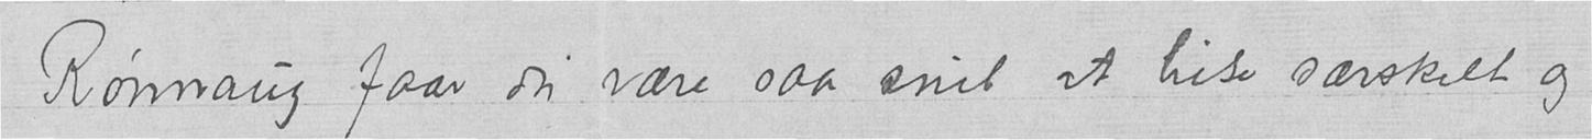Rønnaug faar du være saa snil at hilse særskilt og
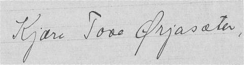Kjære Tore Ørjasæter,
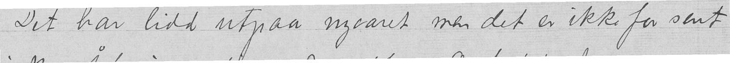Det har lidd utpaa nyaaret, men det er ikke for sent
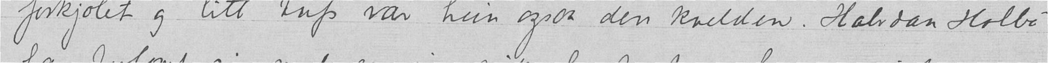forkjølet og litt tufs var hun ogsaa den kvelden. Halvdan Holbø
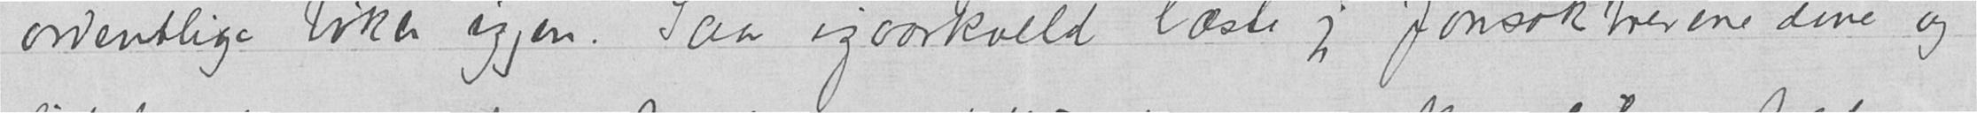ordentlige bøker igjen. Saa igaarkveld læste jeg Jonsokbrevene dine og
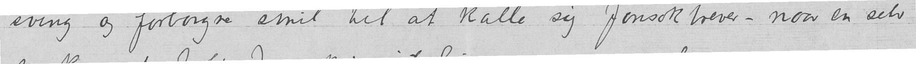sving og forborgne smil til at kalle sig Jonsokbrever – naar en selv
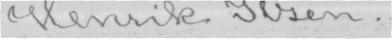Henrik Ibsen.
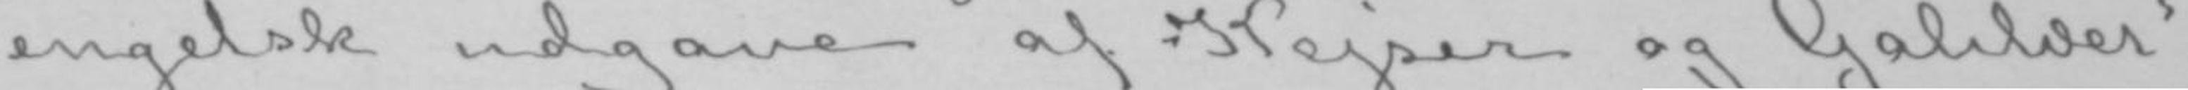engelsk udgave af «Kejser og Galilæer»,
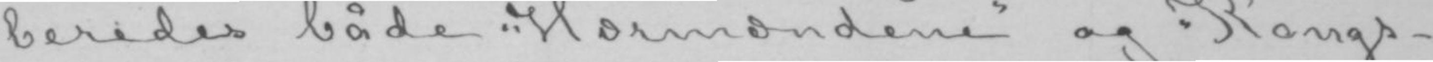beredes både «Hærmændene» og «Kongs-
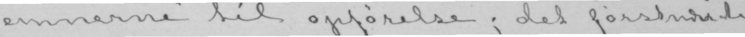emnerne» til opførelse; det førstnævnte
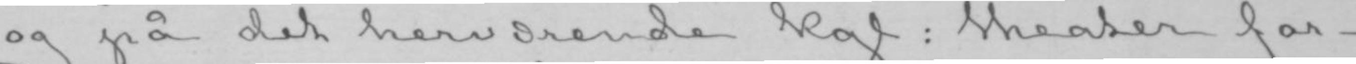og på det herværende kgl: theater for-
